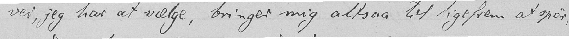vei, jeg har at vælge, bringer mig altsaa til ligefrem at spør-
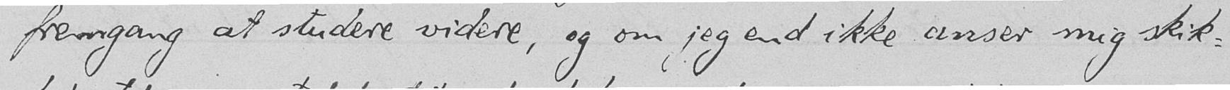fremgang at studere videre, og om, jeg end ikke anser mig skik-
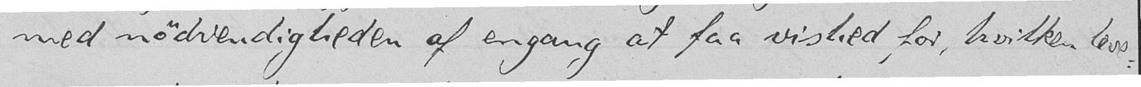med nødvendigheden af engang at faa vished for, hvilken leve-
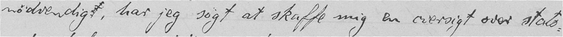nødvendig, har jeg søgt at skaffe mig en oversigt over stats-
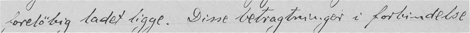foreløbig ladet ligge. Disse betragtninger i forbindelse
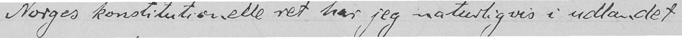Norges konstitutionelle ret har jeg naturligvis i udlandet
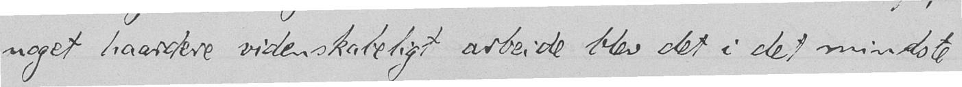noget haardere videnskabeligt arbeide blev det i det mindste
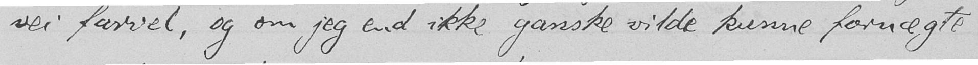vei farvel, og om jeg end ikke ganske vilde kunne fornægte
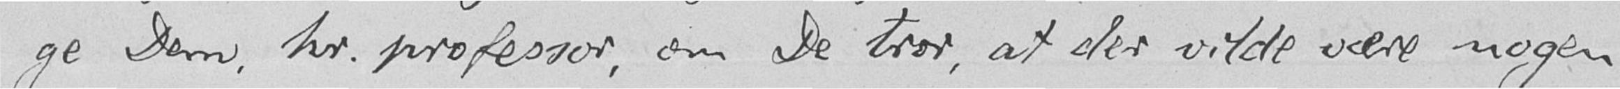ge Dem, hr. professor, om De tror, at det vilde være nogen
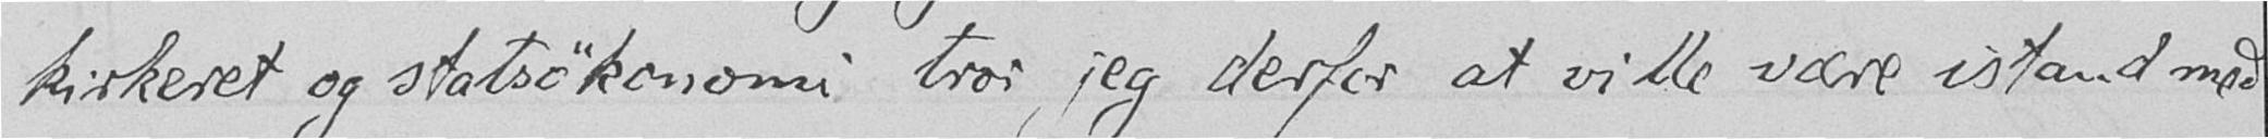kirkeret og statsøkonomi tror jeg derfor at ville være istand med
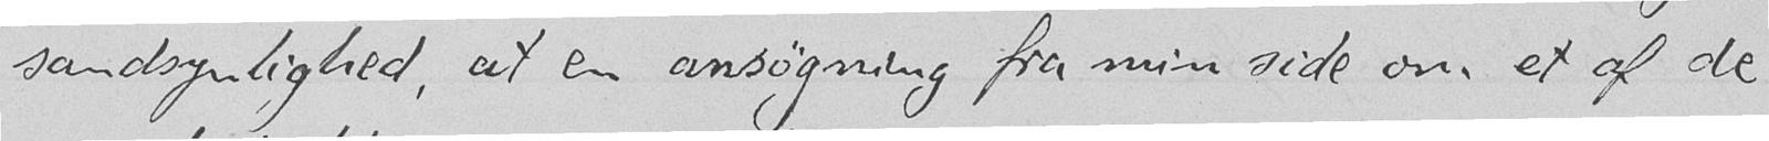sandsynlighed, at en ansøgning fra min side om et af de
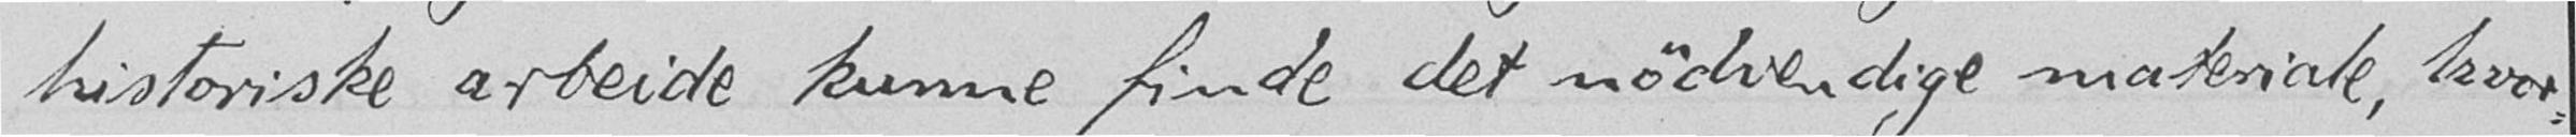historiske arbeide kunne finde det nødvendige materiale, hvor-
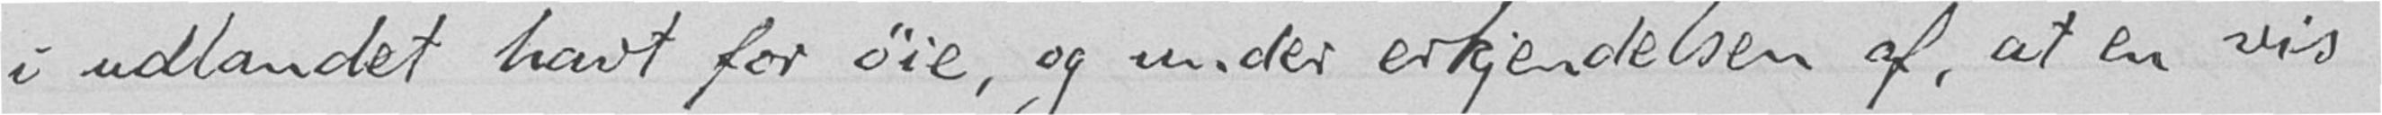i udlandet havt for øie, og under erkjendelsen af, at en vis
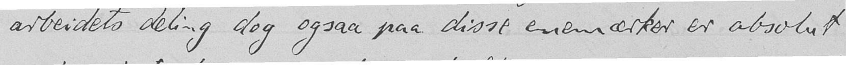arbeidets deling dog ogsaa paa disse enemærker er absolut
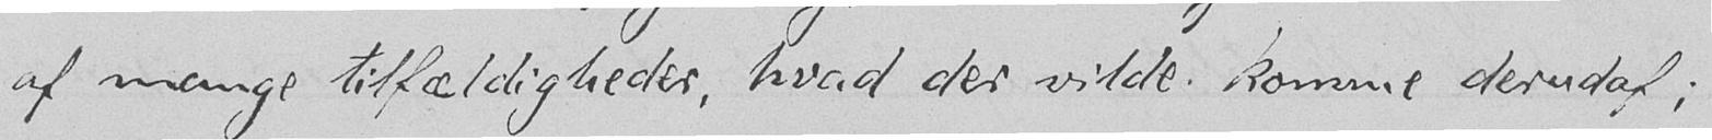af mange tilfældigheder, hvad der vilde komme derudaf;
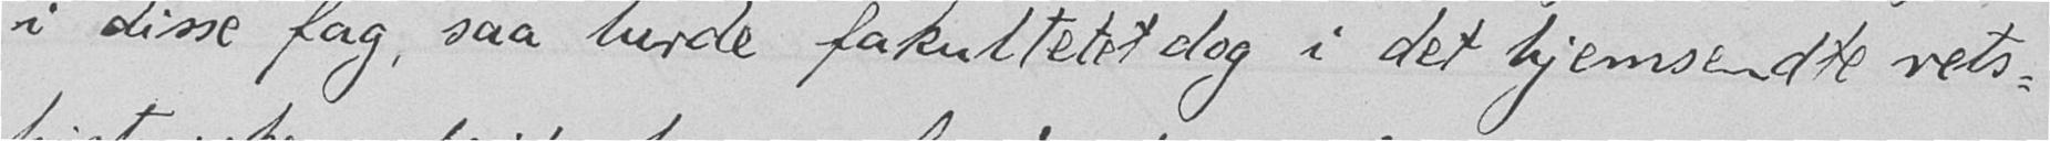i disse fag, saa turde fakultetet dog i det hjemsendte rets-
